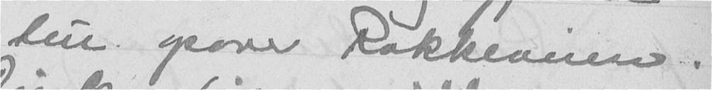tur opover Rokkeveien.
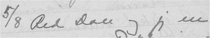5/8 Red Don og jeg en
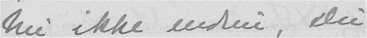Nu, ikke endnu, Du
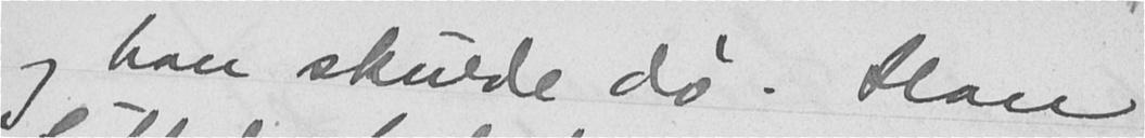og han skulde dø. Han
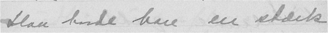Han havde bare en stærk
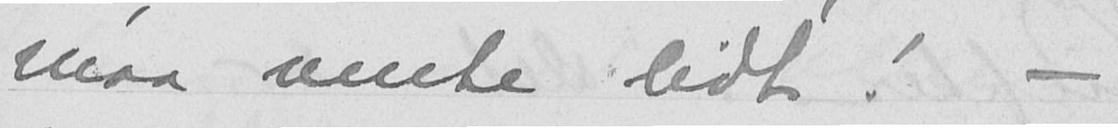maa vente lidt! -
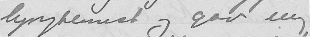lyngblomst og gav mig
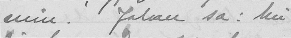min. Johan sa: Nu
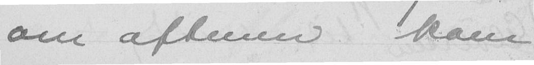om aftenen kom
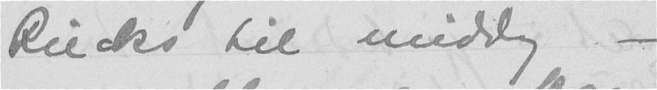Riecks til middag -
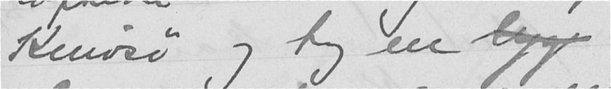Knivsø og tog en lyg
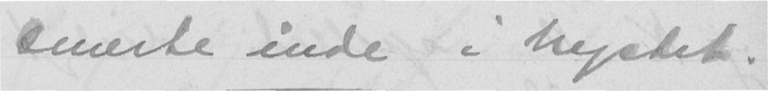smerte inde i brystet.
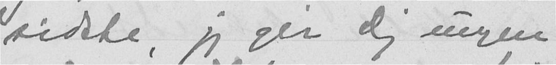sidste jeg gir dig ungen
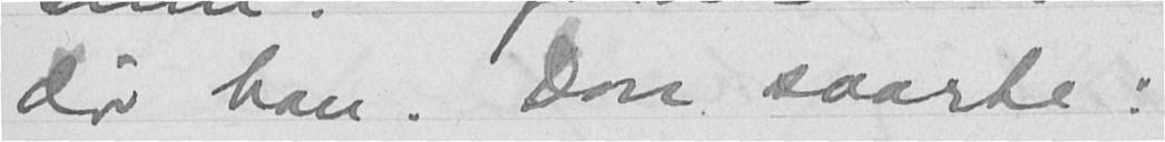dør han. Don svarte:
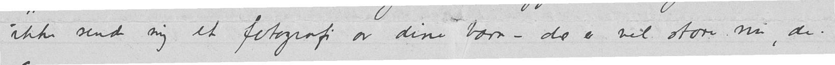ikke sende mig et fotografi av dine barn - de er vel store nu - de.
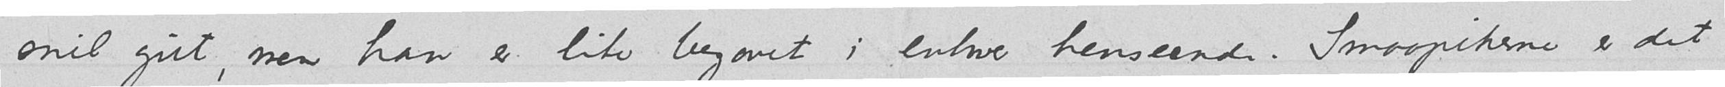snil gut, men han er lite begavet i enhver henseende. Smaapikene er det
